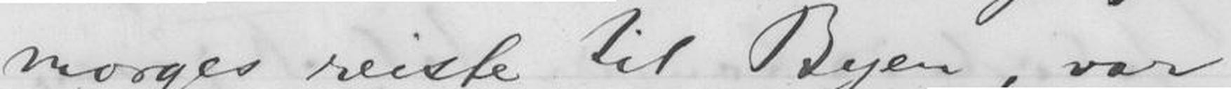morges reiste til Byen, var
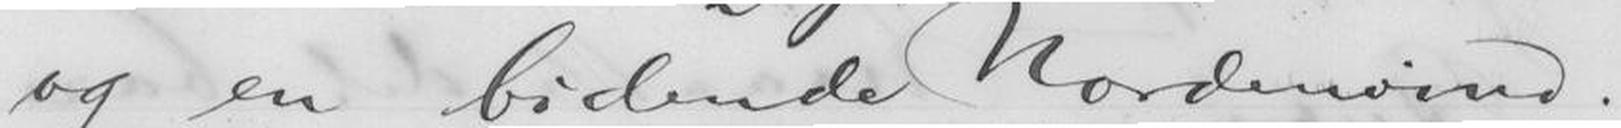og en bidende Nordenvind.
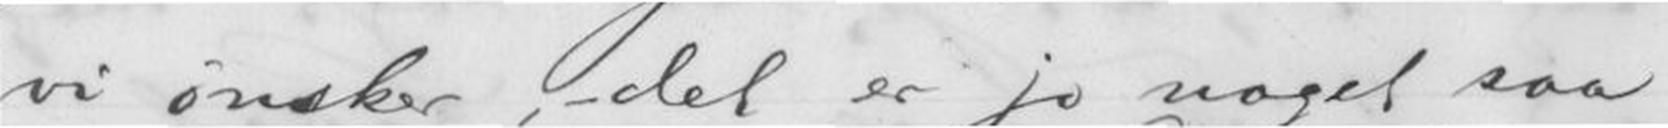vi ønsker, - det er jo noget saa
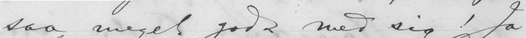saa meget godt med sig! Ja
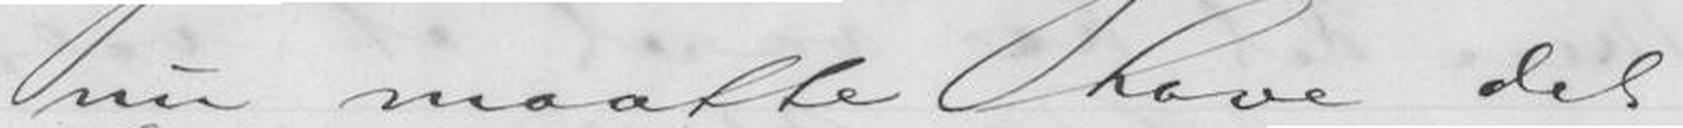nu maatte have det
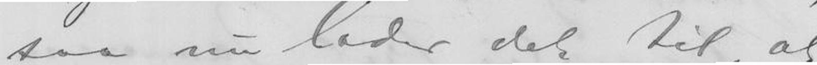saa nu lader det til, at
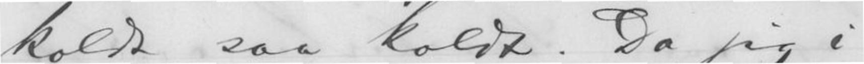koldt saa koldt. Da jeg i-
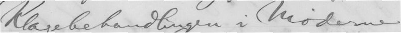Klagebehandlingen i Møderne,
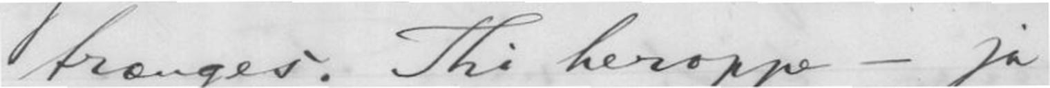trænges. Thi heroppe - ja
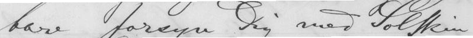bare forsyn Dig med Solskin
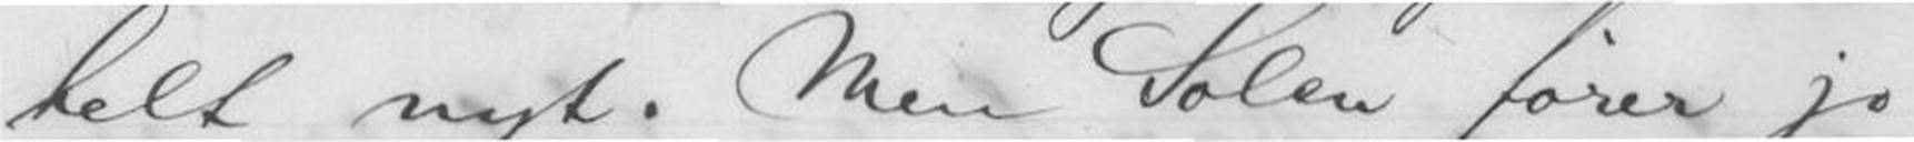helt nyt. Men Solen fører jo
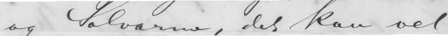og Solvarme, det kan vel
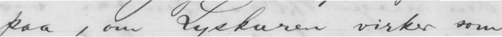paa, om Lyskuren virker som
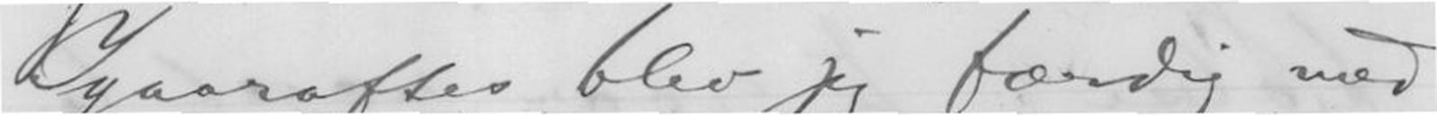Igaaraftes blev jeg færdig med
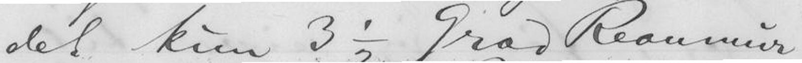det kun 3 ½ Grad Reaumur
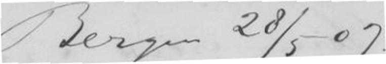Bergen 28/5 07.
